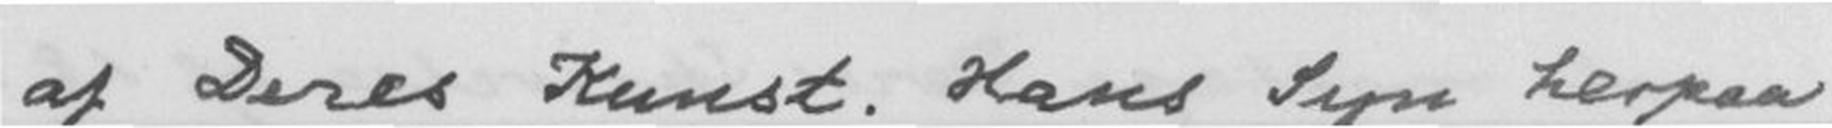af Deres Kunst. Hans Syn herpaa
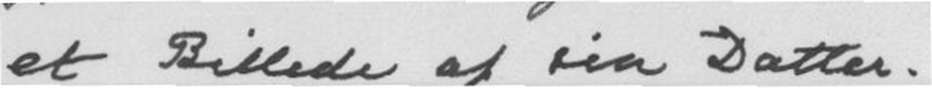et Billede af sin Datter.
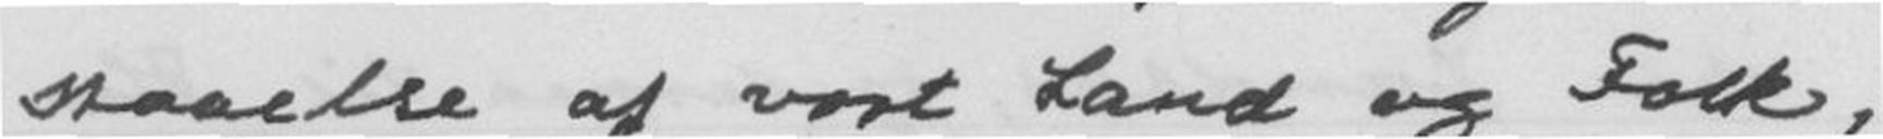staalse af vort Land og Folk,
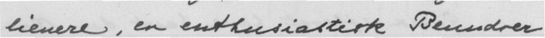lienere, en enthusiastisk Beundrer
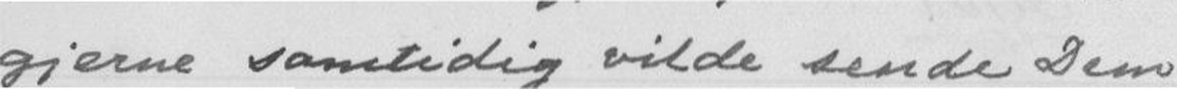gjerne samtidig vilde sende Dem
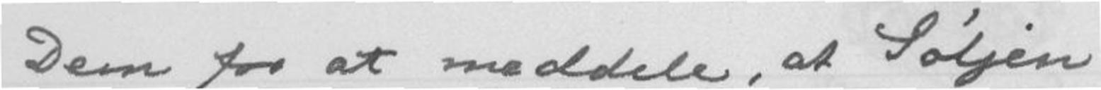Dem for at meddele, at Søljen
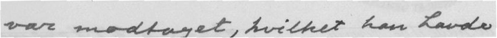var modtaget, hvilket han havde
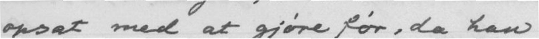opsat med at gjøre før, da han
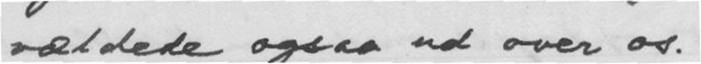vældede ogsaa ud over os.
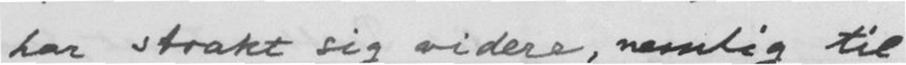har strakt sig videre, nemlig til
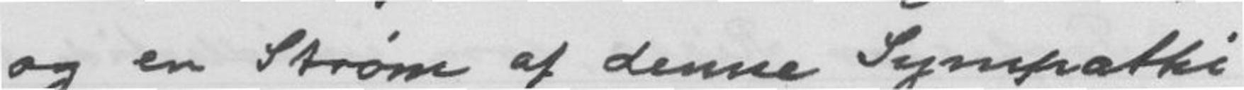og en Større af denne Sympathi
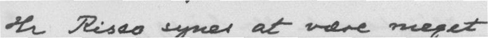Hr Risso synes at være meget
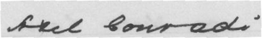Axel Conradi
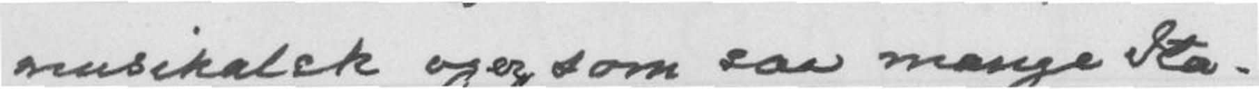musikalsk og er, som saa mange Ita-
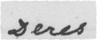Deres
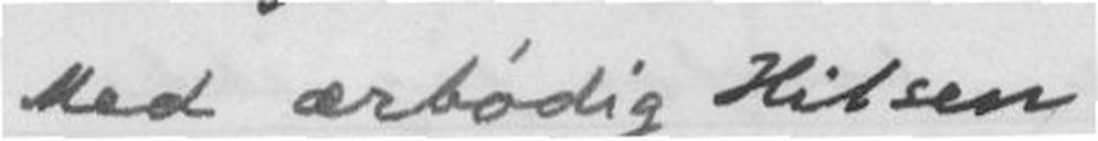Med ærbødig Hilsen
